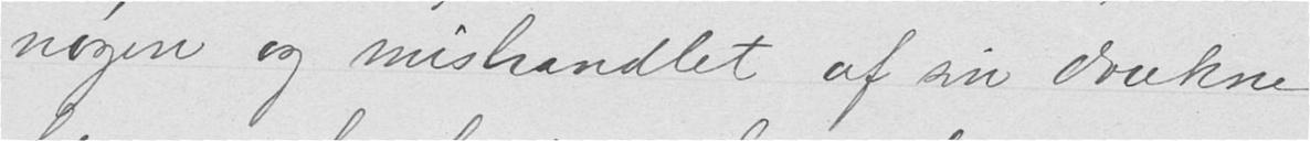nøgen og mishandlet af sin drukne
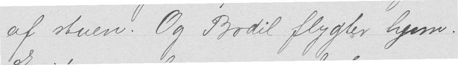af stuen. Og Bodil flygter hjem.
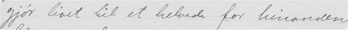gjør livet til et helvede for hinanden.
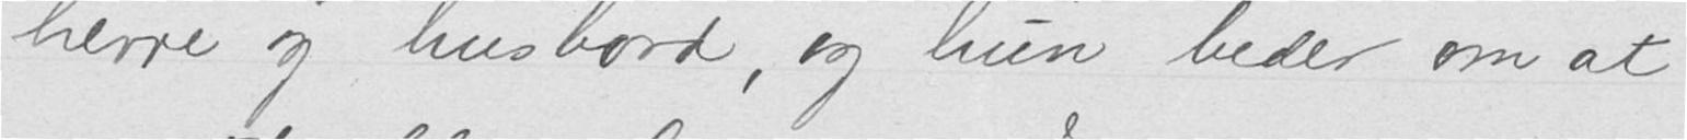herre og husbond, og hun beder om at
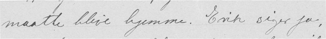maatte blive hjemme. Erik siger ja,
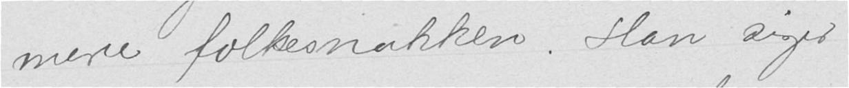mere folkesnakken. Han siger
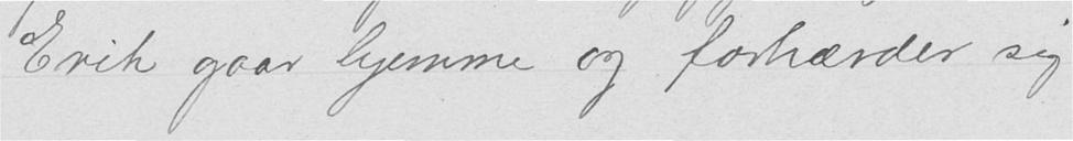Erik gaar hjemme og forhærder sig
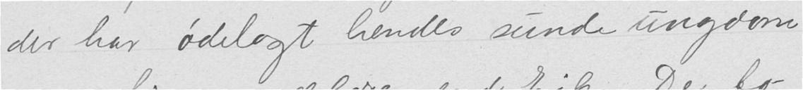der har ødelagt hendes sunde ungdom
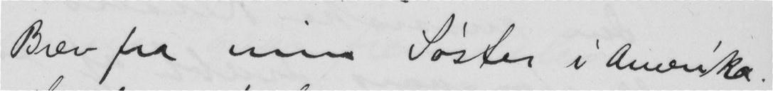Brev fra min Søster i Amerika.
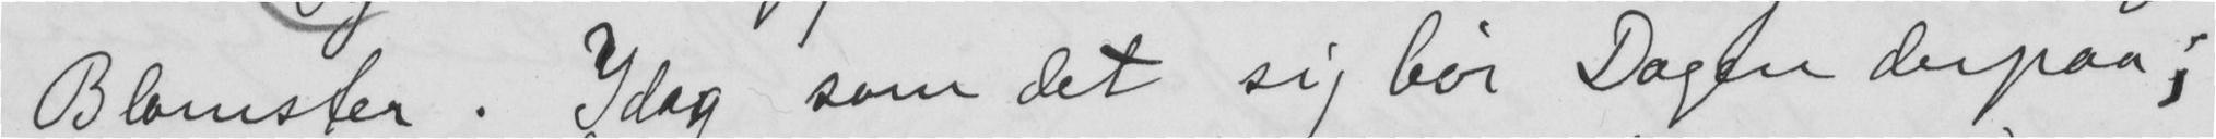Blomster. Idag som det sig bør Dagen derpaa;
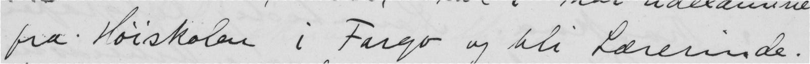fra Høiskolen i Fargo og bli Lærerinde.
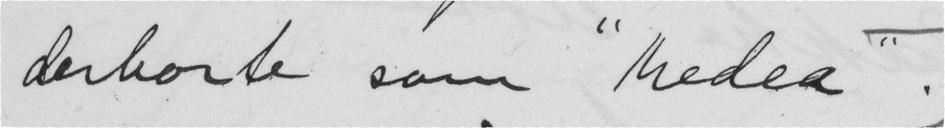derborte som "Medea".
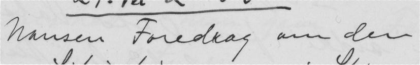Nansen Foredrag om den
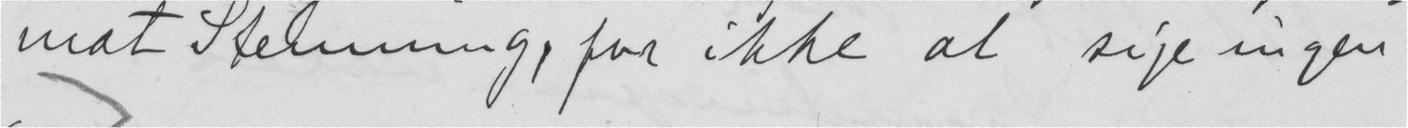mat Stemning, for ikke at sige ingen
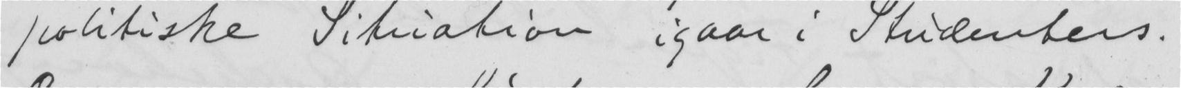politiske Situation igaar i Studenters.
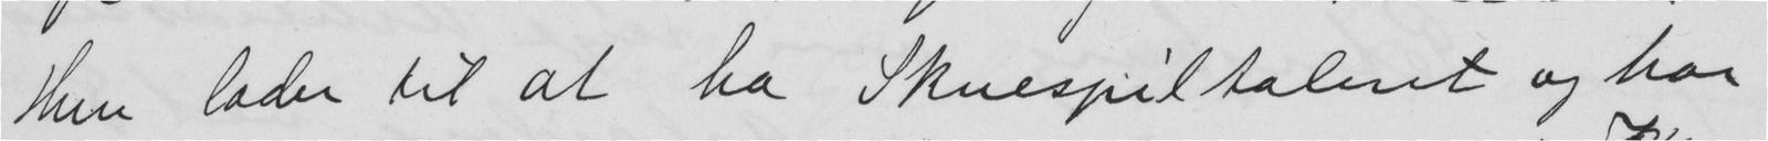Hun lader til at ha Skuespiltalent og har
